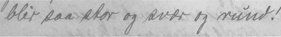blir saa stor og svær og rund!
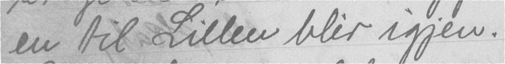en til Lillen blir igjen.
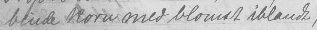binde korn med blomst iblandt,
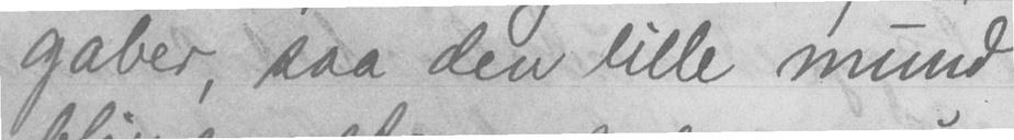gaber, saa den lille mund
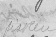fisken
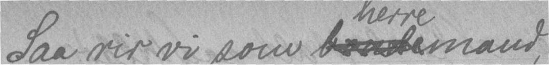Saa rir vi som herremand,
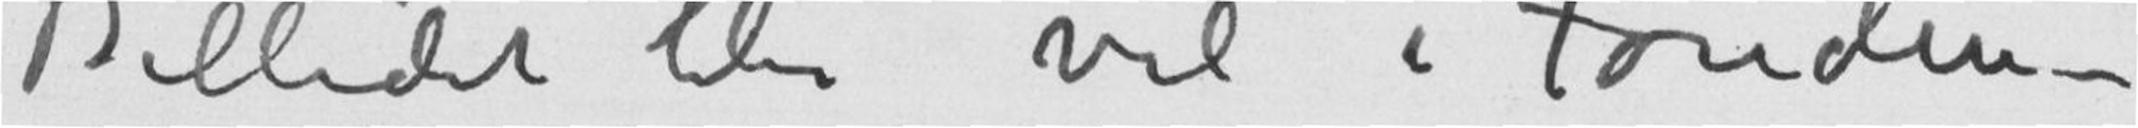Billedet blir vel i Fonden –
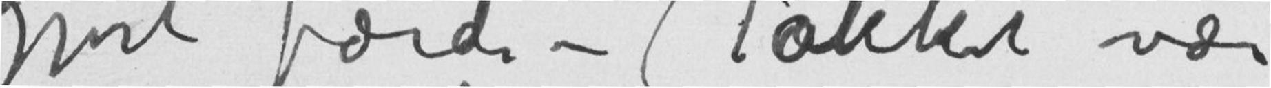gjort fordi – (Takket være
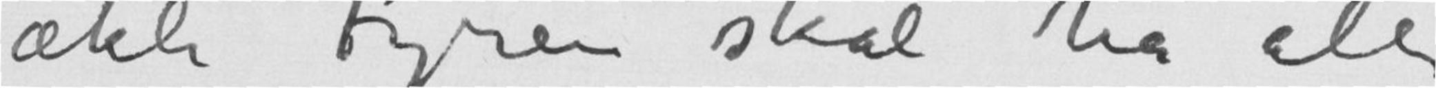ækle Fyren skal ha alle
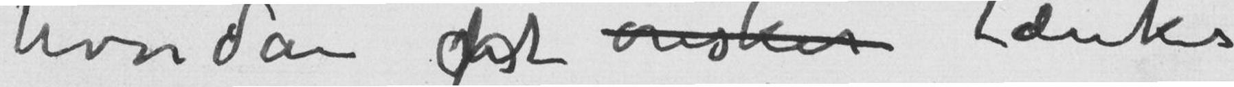hvordan det ønsker tænkes
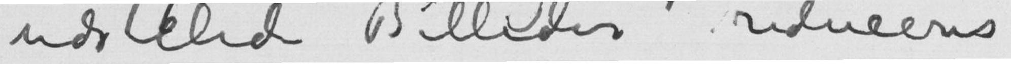udstillede Billeder reduceres
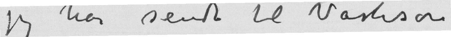jeg har sendt til Vasteson
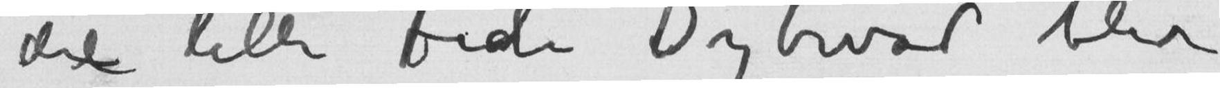den lille fede Dybwad blir
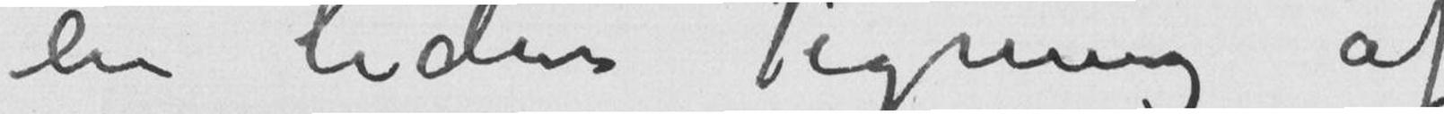en liden Tegning af
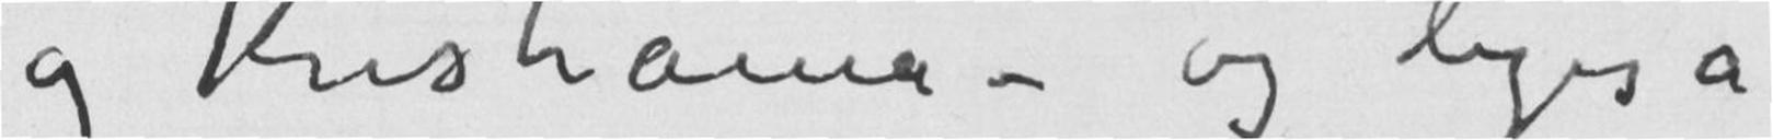og Kristiania – og ligeså
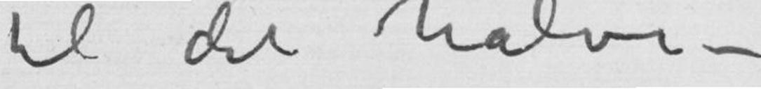til det halve –
